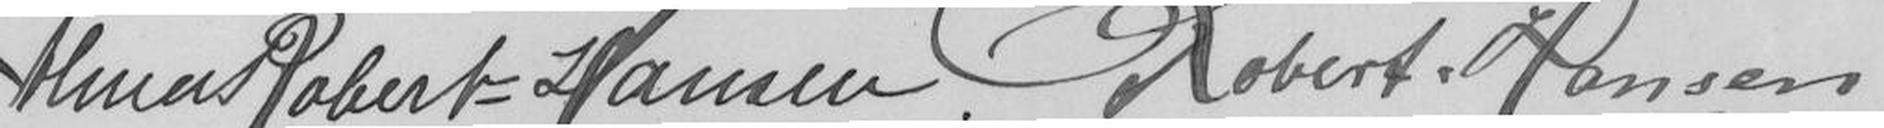Almers Robert=Hansen Robert=Hansen
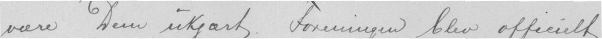være Dem ukjært. Foreningen blev officielt
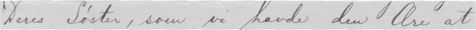Deres Søster, som vi havde den Ære at
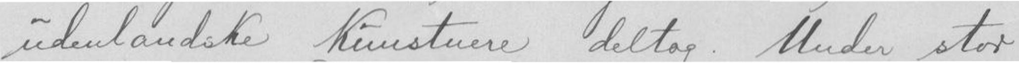udenlandske Kunstnere deltog. Under stor
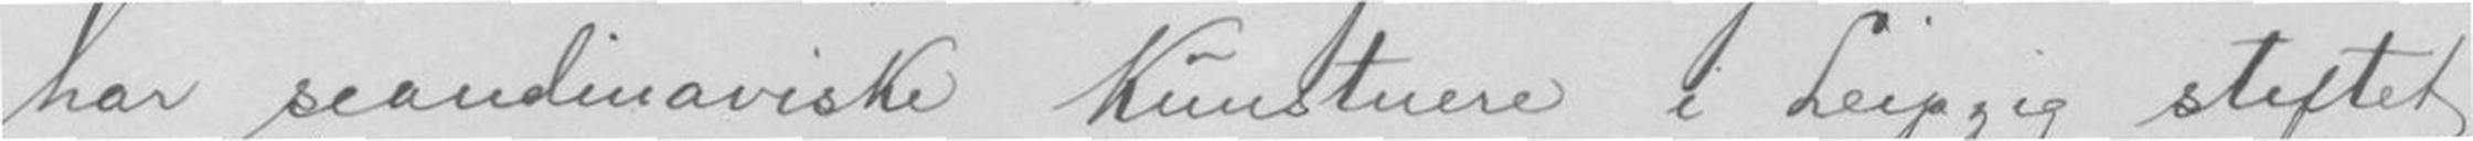har scandinaviske Kunstnere i Leipgig stiftet
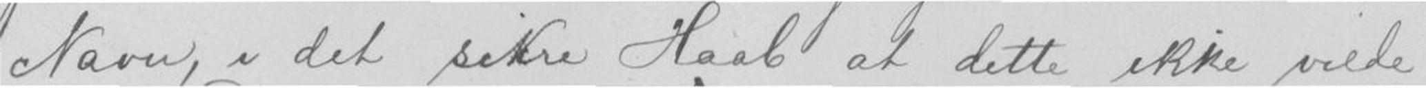Navn, i det sikre Haab at dette ikke vilde
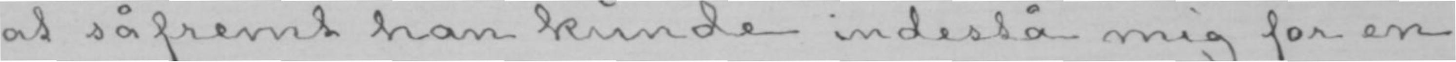at såfremt han kunde indestå mig for en
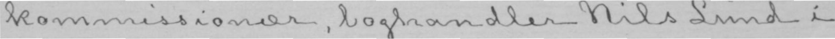kommissionær, boghandler Nils Lund i
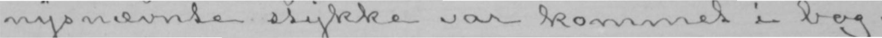nysnævnte stykke var kommet i bog-
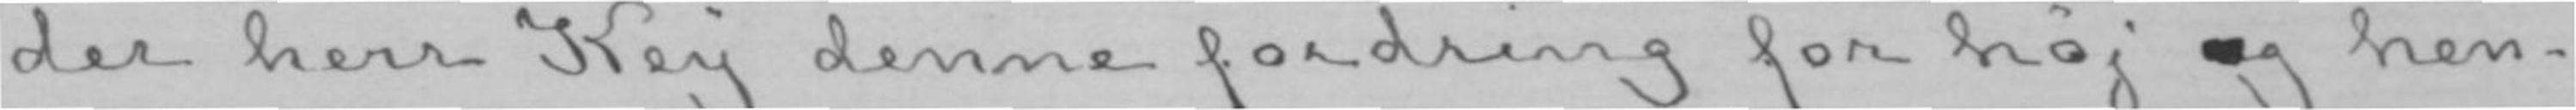der herr Key denne fordring for høj og hen-
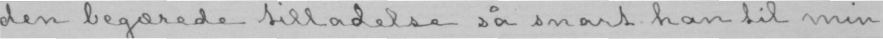den begærede tilladelse så snart han til min
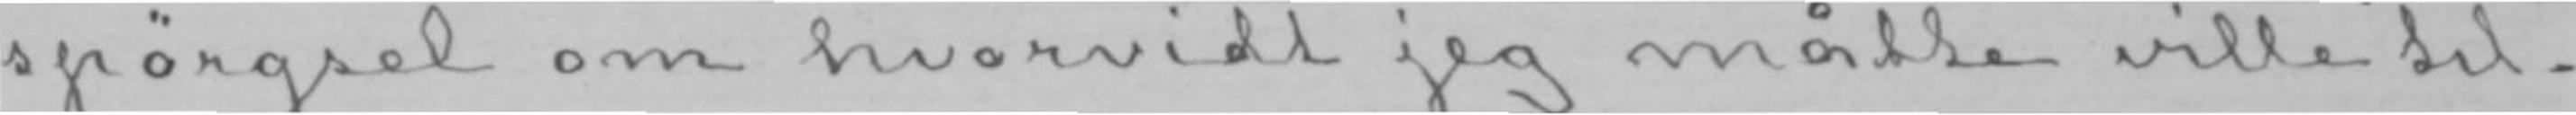spørgsel om hvorvidt jeg måtte ville til-
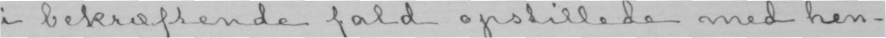i bekræftende fald opstillede med hen-
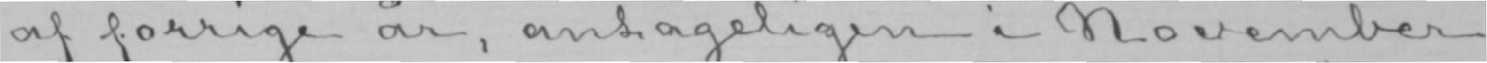af forrige år, antageligen i November
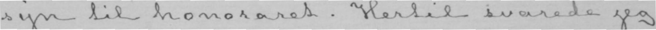syn til honoraret. Hertil svarede jeg
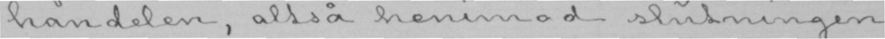handelen, altså henimod slutningen
